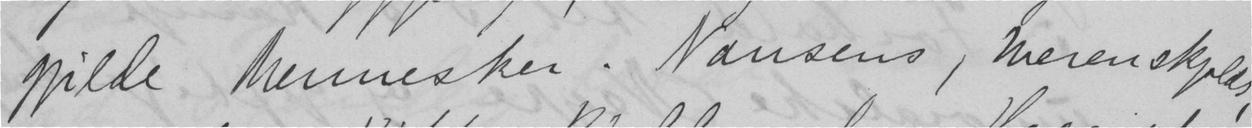gjilde mennesker. Nansens, Werenskjolds,
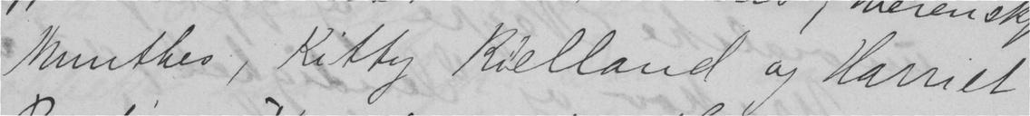Munthes, Kitty Kielland og Harriet
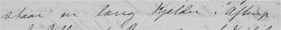staar en lang Kjælder i "Aftenp."
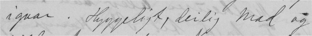igaar. Hyggeligt,deilig mad og
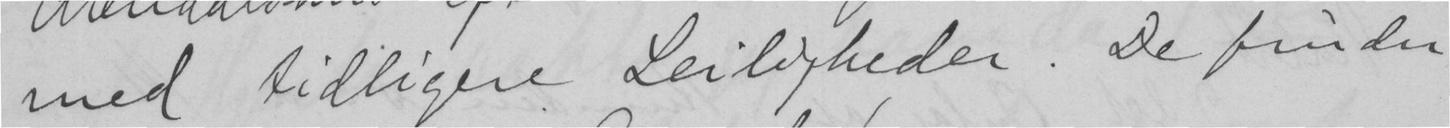med tidligere Leiligheder. De finder
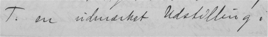T. en udmærket Udstilling i
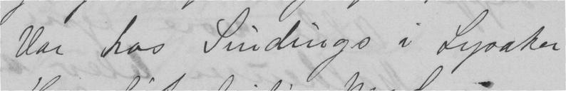Var hos Sindings i Lysaker
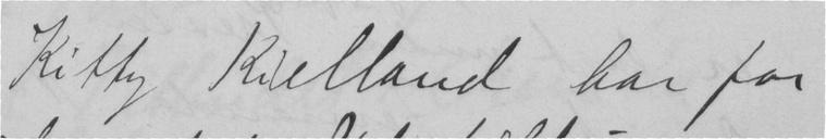Kitty Kielland har for
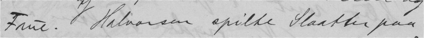Frue. Halvorsen spilte Slaatter paa
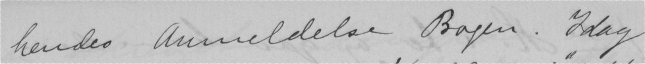hendes Anmeldelse Bogen. Idag
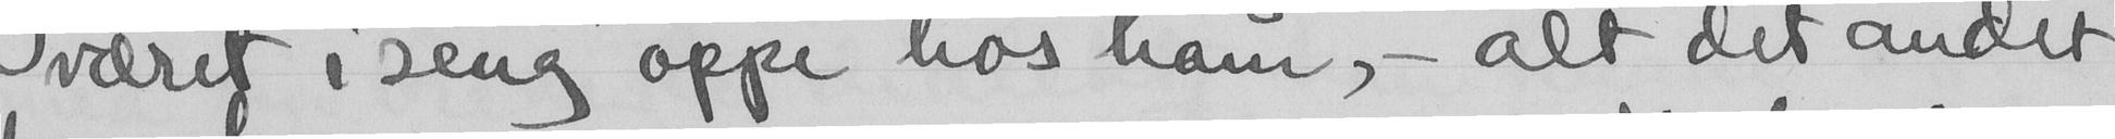været i seng oppe hos ham, - alt det andet
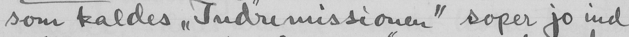som kaldes "Indremissionen" soper jo ind
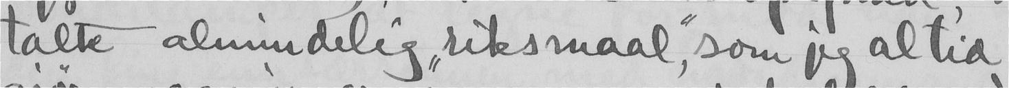talte almindelig "riksmaal", som jeg altid
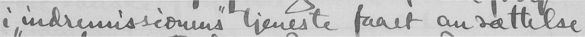i "indremissionens" tjeneste faaet ansættelse
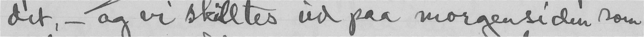det, - og vi skilltes ud paa morgensiden som
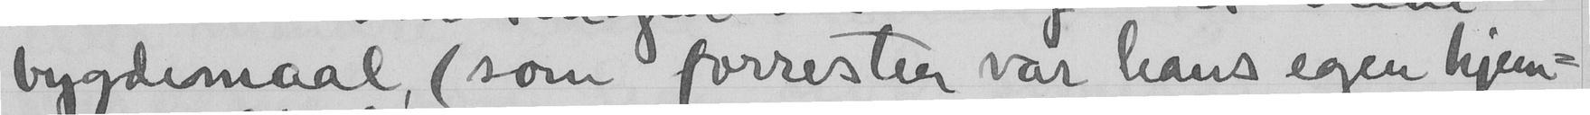bygdemaal, (som forresten var hans egen hjem-
